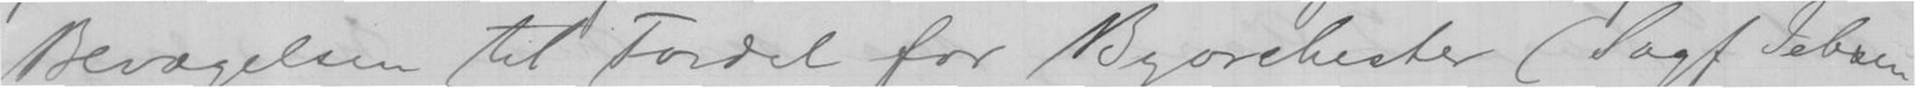Bevægelsen til Fordel for Byorchester (Sagf Jebsen,
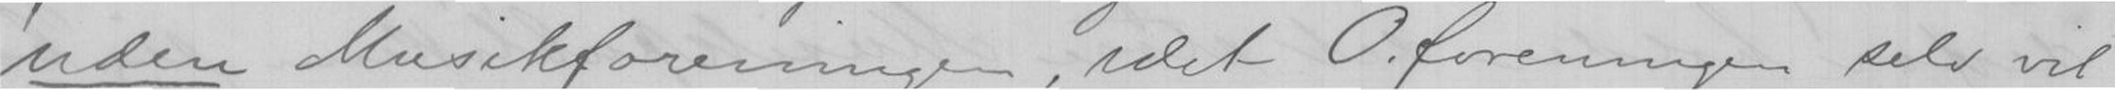uden Musikforeningen, idat O.foreningen selv vil
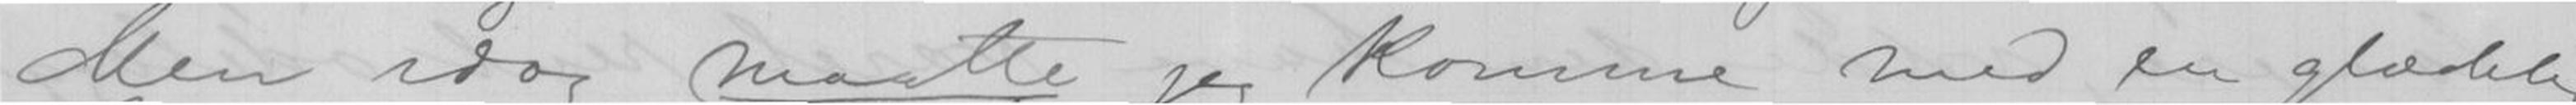Men idag maatte jeg komme med en glædelig
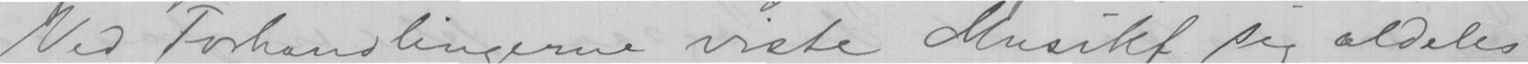Ved Forhandlinger viste Musikf sig aldeles
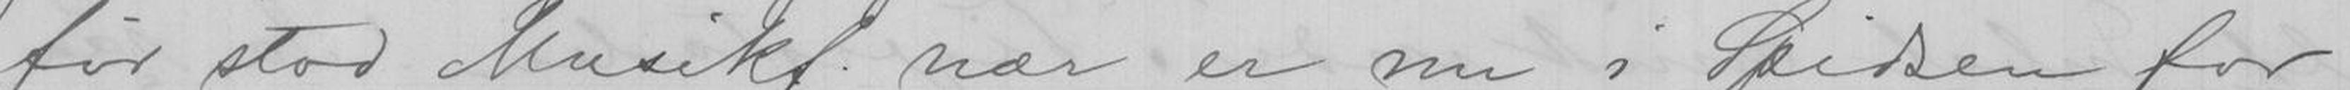før stod Musikf. nær er nu i Spidsen for
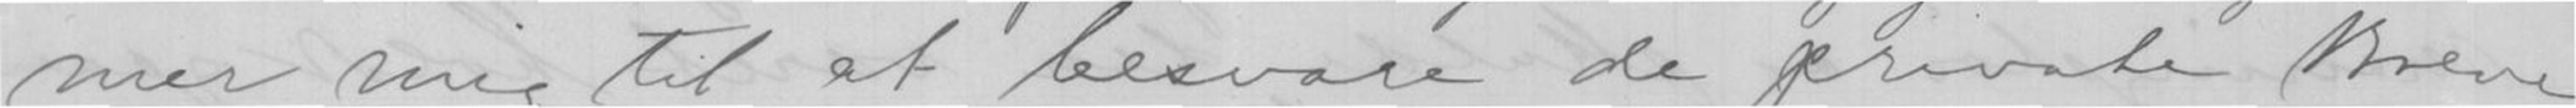mer mig til at besvare de private Breve
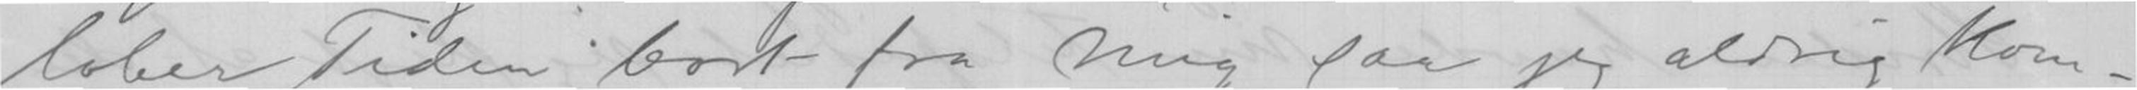løber Tiden bortt fra mig saa jeg aldrig kom-
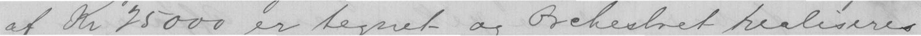af Kr 25000 er tegnet og Orchestret realiseres
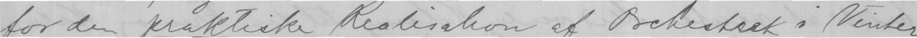for den praktiske Realisation af Orchestret i Vinter
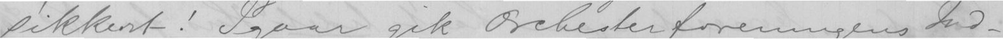sikkert! Igaar gik Orchesterforeningens Ind-
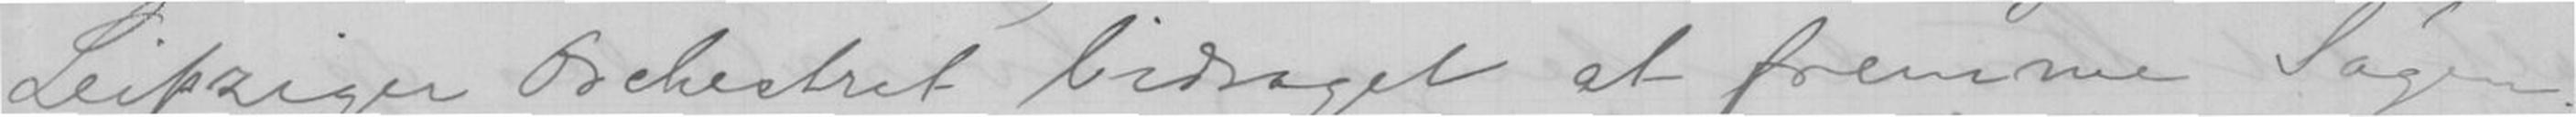Leipziger Orchestret bidraget at fremme Sagen.
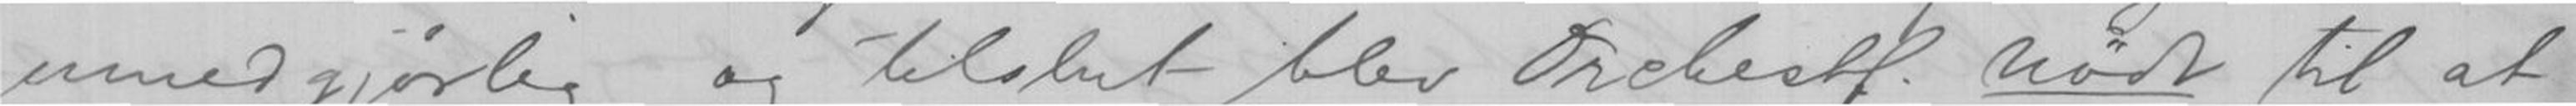umedgjørlig og tilslut blev Orchestf. nødt til at
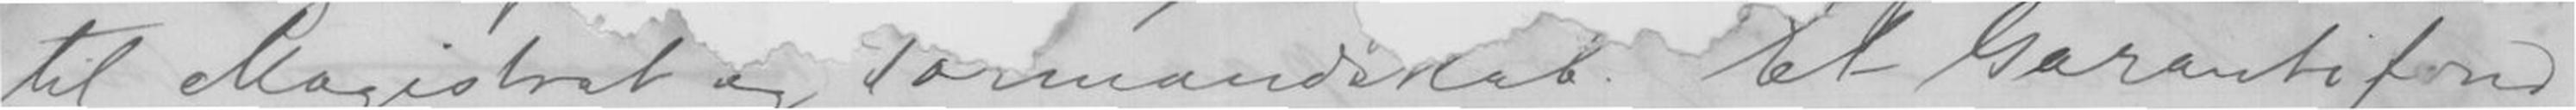til Magistrat og Formandskab. Et Garantifond
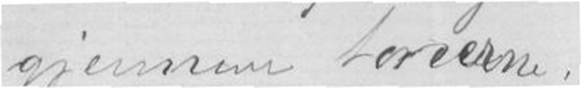gjennem tonerne.
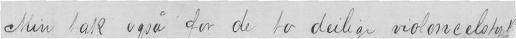Min tak også for de to deilige violoncelstykker
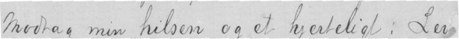Modtag min hilsen og et hjerteligt: Lev
